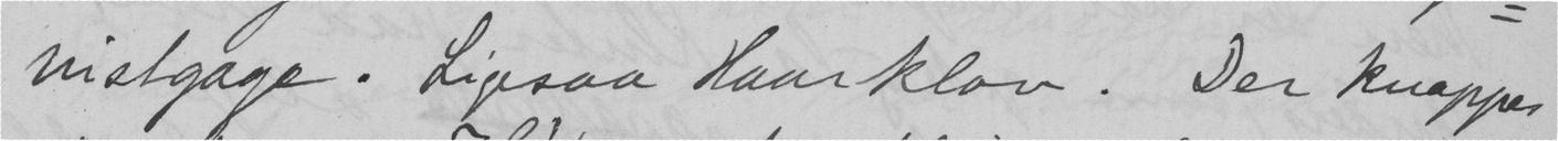nistgage. Ligesaa Haarklou. Der knappes
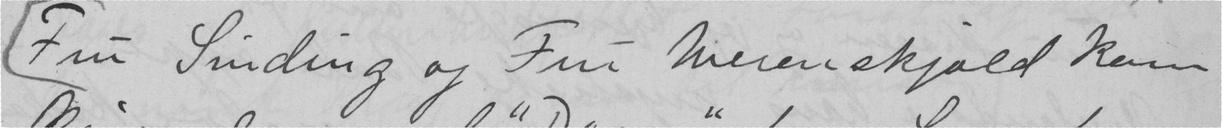Fru Sinding og Fru Werenskjold kom
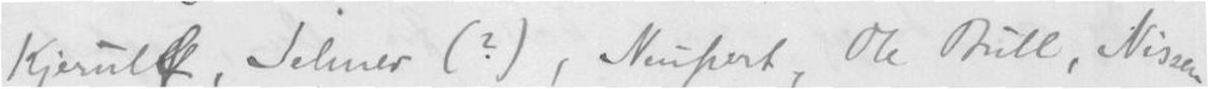Kjerulf, Sehmer (?), Neupert, Ole Bull, Nissen
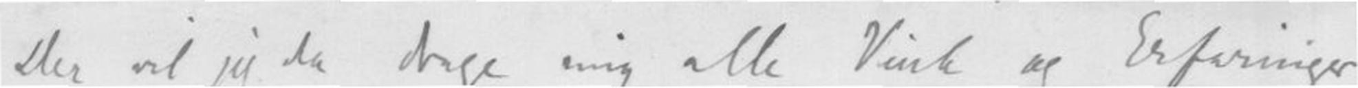Der vil jeg da tage mig alle Vink og Erfaringer
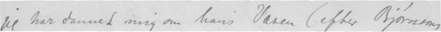jeg har dannet mig om hans Væren (efter Bjørnsons
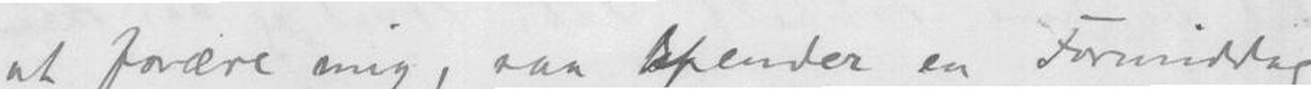at forære mig, saa spender en Formiddag
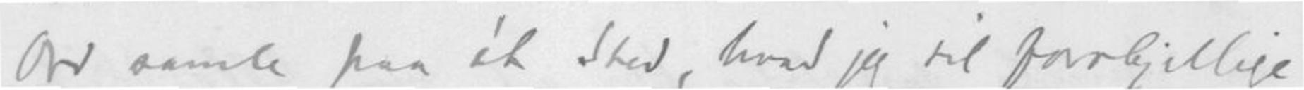Ord samle paa it Sted, hvad jeg til forskjellige
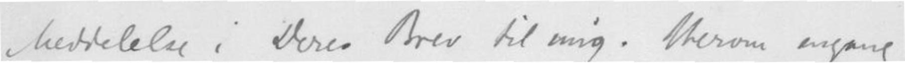berretelse i Deres Brev til mig. Herom engang
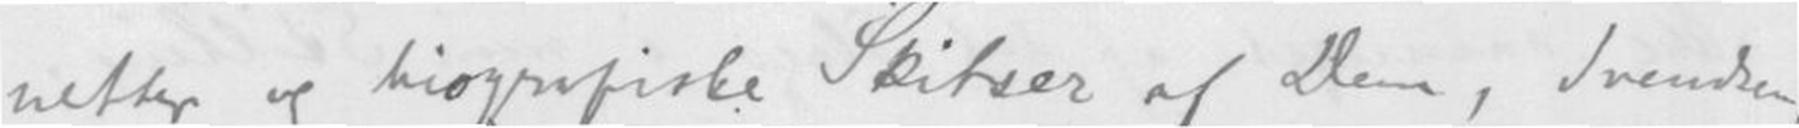netter og biografiske Skitser af Dem, Svendsen,
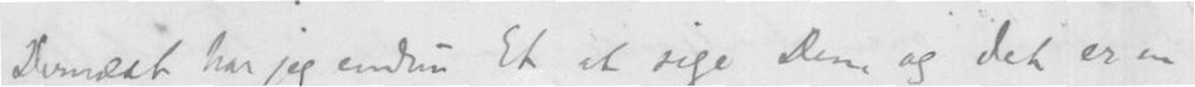Dernæst har jeg endnu Et at sige Dem, og det er en
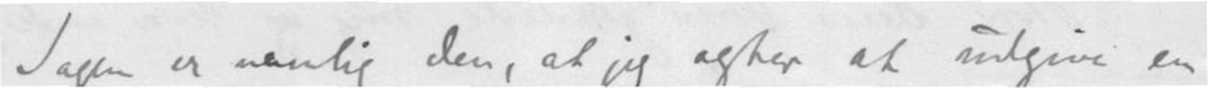Sagen er sanlig den, at jeg agter at udgive en
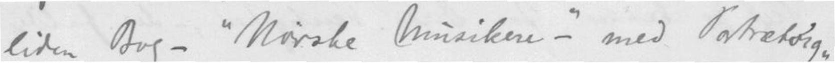ligen Bog - "Norske Musikere-" med Portrætvig-
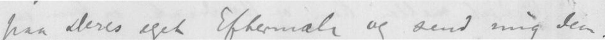paa Deres eget Eftermæl, og send mig dem.
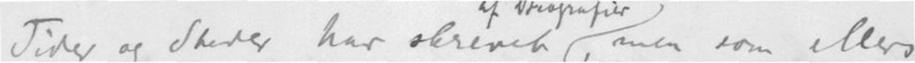Tider og Stunder har skrevet af, men som ellers
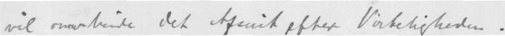vil omarbeide det Afsnitt efter Virkeligheden
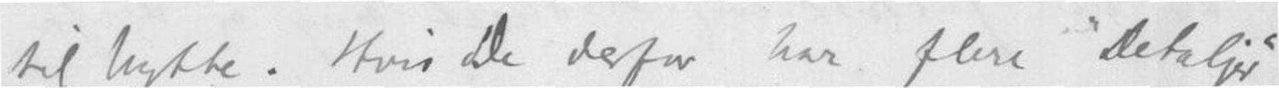til bytte. Hvis De derfor har flere "Detaljer"
